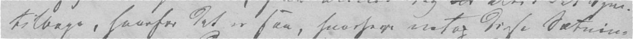tilbage, hvorfor det er saa, hvorfor netop disse Sætnin-
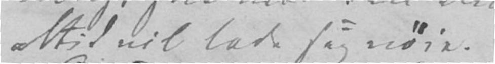altid vil lade sig nøie.
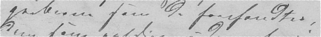greberne som de forefandtes,
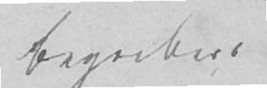Begrebers
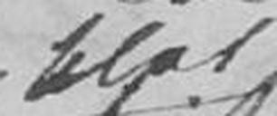blot
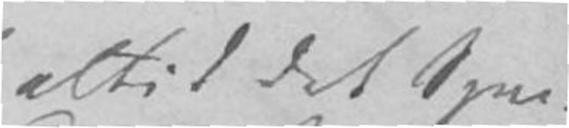altid det Spm.
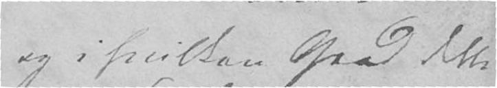og i hvilken Grad dette
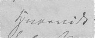Hvorvidt
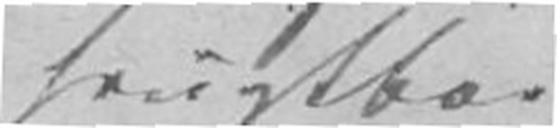frugtbar
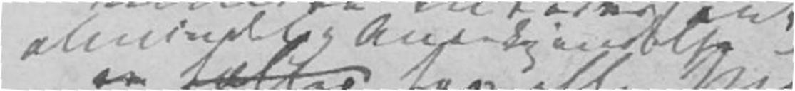almindelig Anerkjendelse
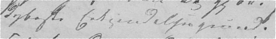dybeste Erkjendelsesgrunde.
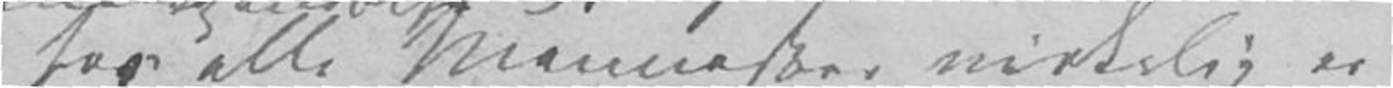hos alle Mennesker virkelig er
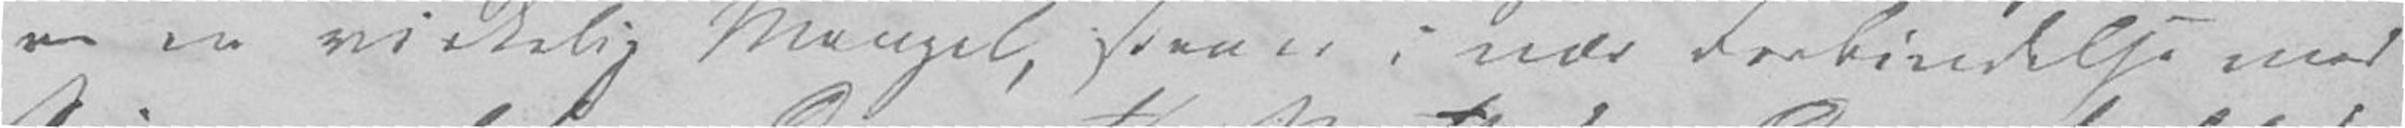er en virkelig Mangel, staaer i nær Forbindelse med
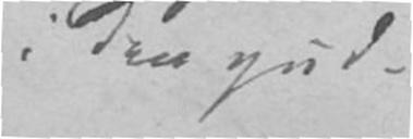i den gud-
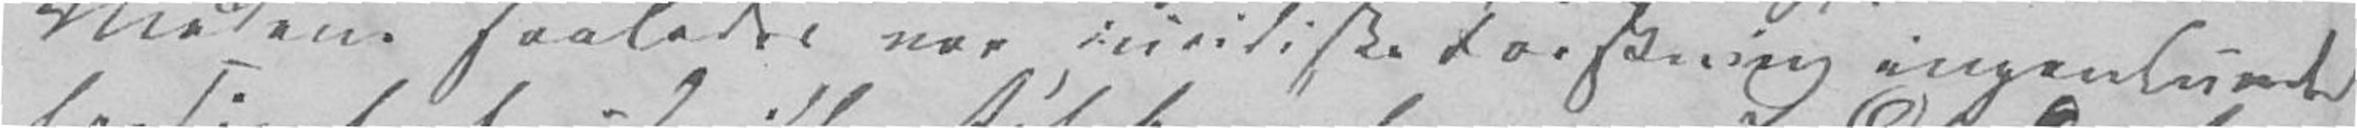Medens saaledes vor juridiske Forskning ingenlunde
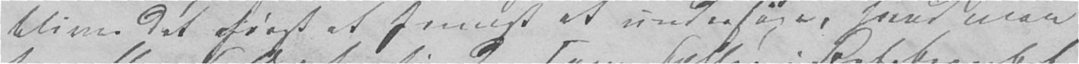bliver det først et frugt at undersøge, hvad man
